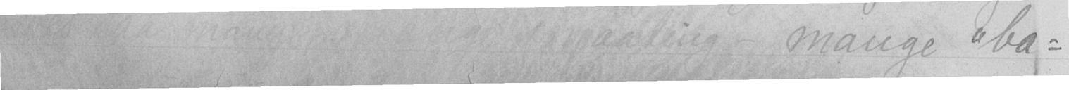ves saa mange  smaating- mange "ba-
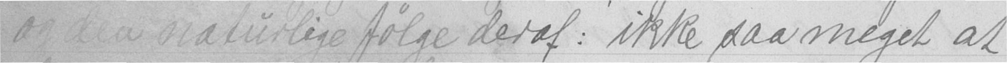og den naturlige følge deraf: ikke saa meget at
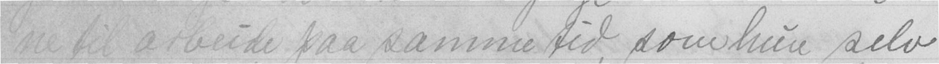ne til arbeide paa samme tid, som hun selv
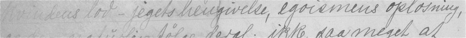kvindens lod - jegets hengivelse, egoismens opløsning,
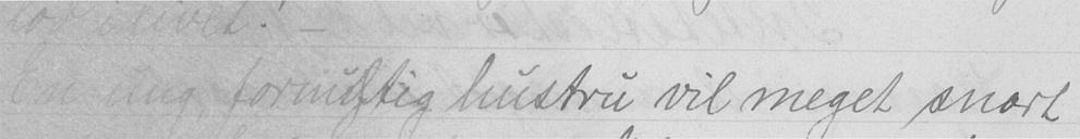En ung, fornuftig hustru vil meget snart
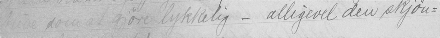blive som at gjøre lykkelig - alligevel den skjøn-
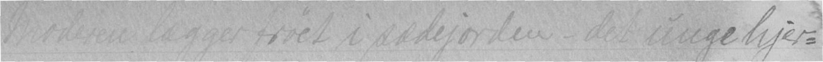Moderen lægger frøet i sædejorden - det unge hjert-
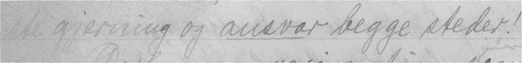ste gjerning og ansvar begge steder!
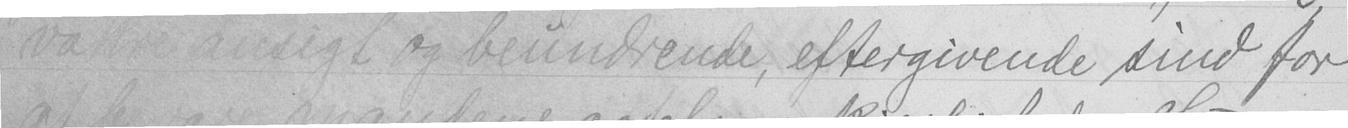vakre ansigt og beundrende, eftergivende sind for
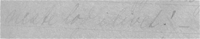neste lod i livet! -
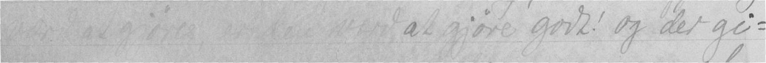værd at gjøre, er den værd at gjøre godt! og der gi-
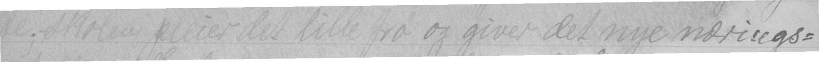te; skolen pleier det lille frø og giver det nye nærings-
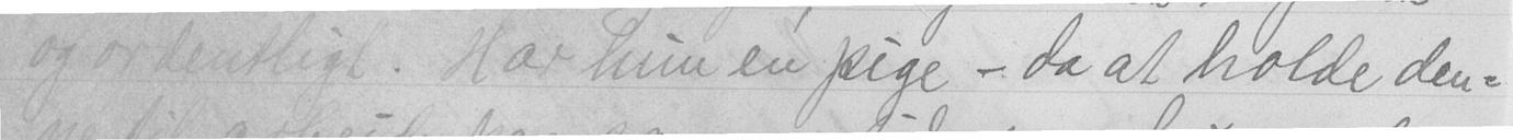og ordentligt. Har hun en pige - da at holde den-
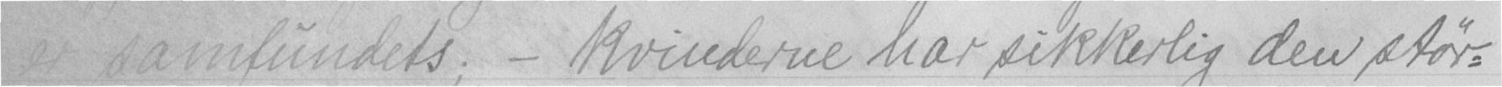er samfundets; - kvinderne har sikkerlig den stør-
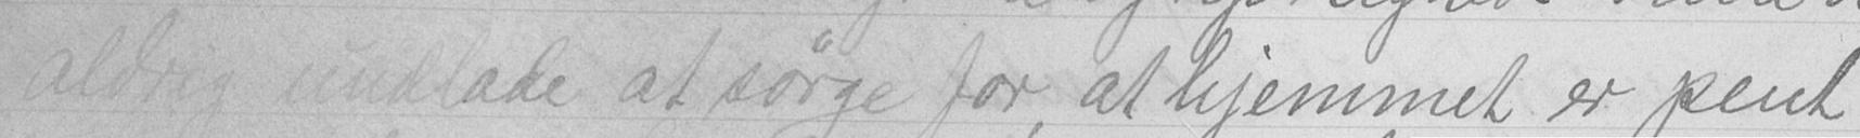aldrig undlade at sørge for at hjemmet er pent
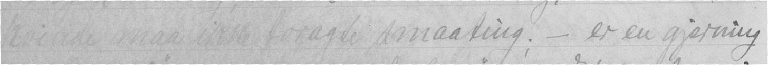kvinde maa ikke foragte smaating; - er en gjerning
